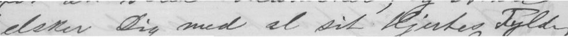elsker Dig med al sit Hjertes Fylde,
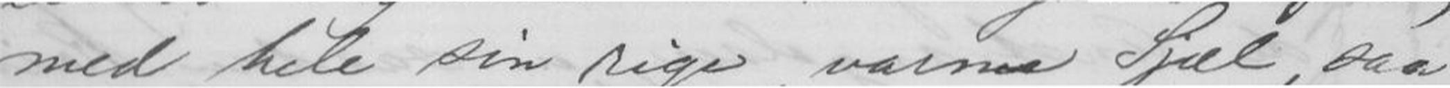med hele sin rige, varme Sjæl, saa
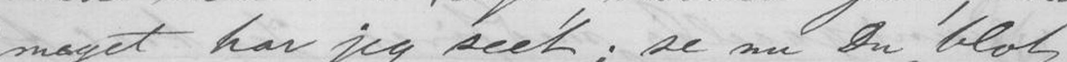meget har jeg seet; se nu Du blot
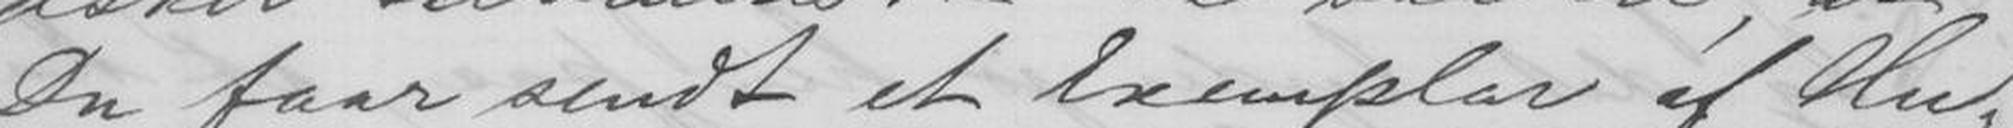Du faar sendt et Exemplar af Hu-
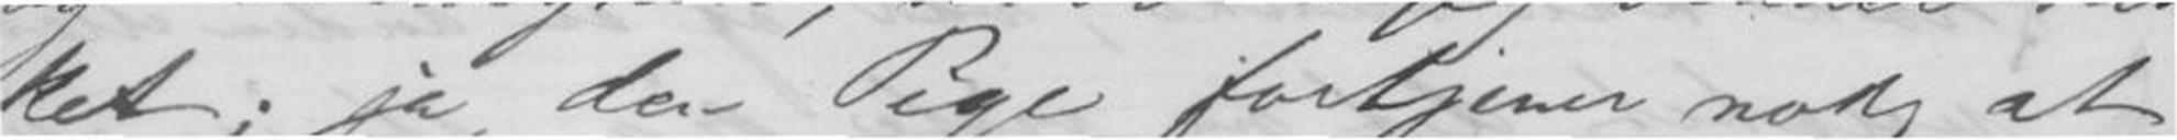ket; ja, den Pige fortjener nok, at
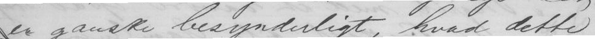er ganske besynderligt, hvad dette
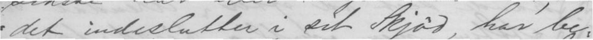det indeslutter i sit Skjød, har be-
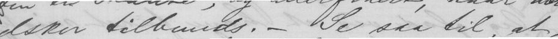elsker tilbunds. - Se saa til, at
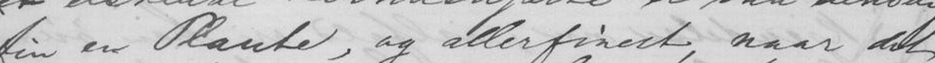fin en Plante, og allerførst, naar det
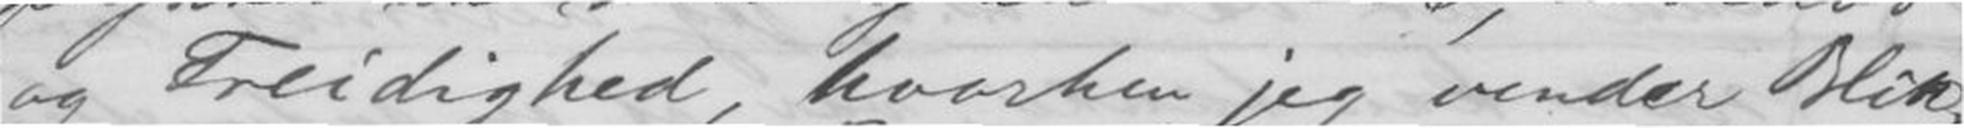og Freidighed, hvorhen jeg vender Blik-
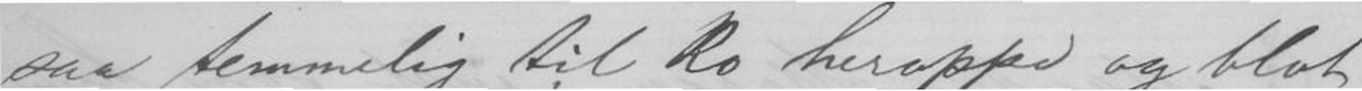saa temmelig til Ro heroppe og blot
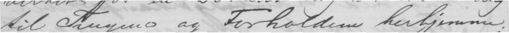til Tingene og Forholdene herhjemme;
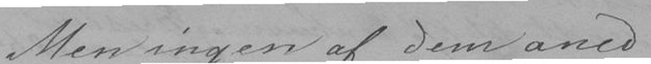Men ingen af dem aned
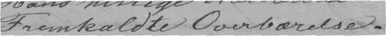Fremkaldte Overbærelse.
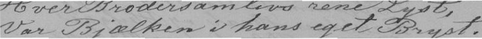Var Bjælken i hans eget Bryst.
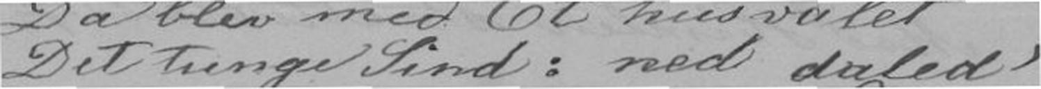Det tunge Sind; ned daled'
